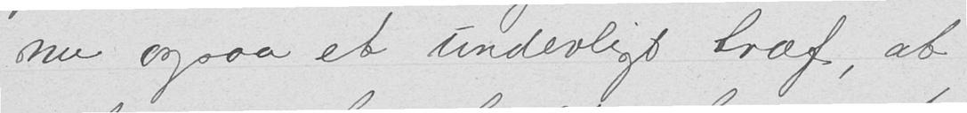nu ogsaa et underligt træf, at
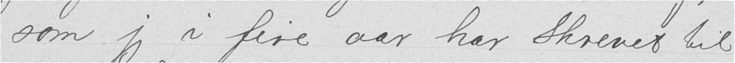som jeg i fire aar har skrevet til
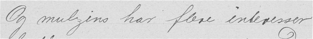Og muligens har flere interesser
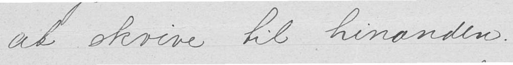at skrive til hinanden.
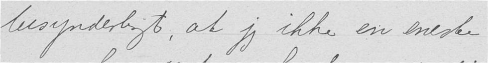besynderligt, at jeg ikke en eneste
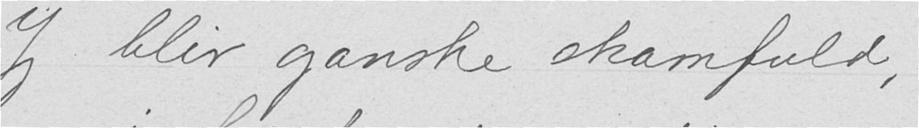Jeg blir ganske skamfuld,
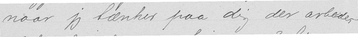naar jeg tænker paa dig der arbeider
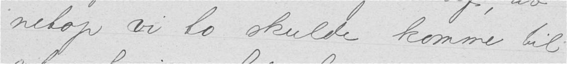netop vi to skulde komme til
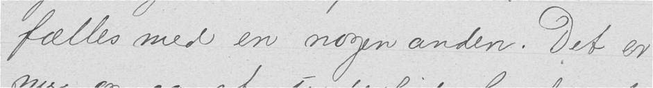fælles med en nogen anden. Det er
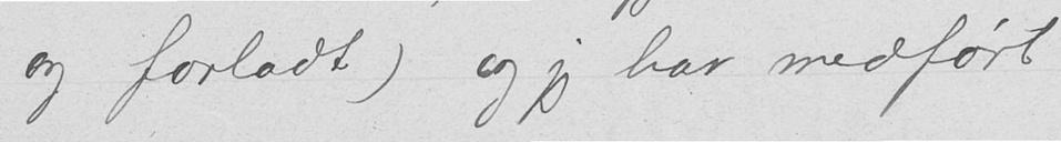og forladt) og jeg har medført
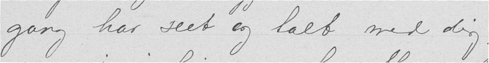gang har seet og talt med dig,
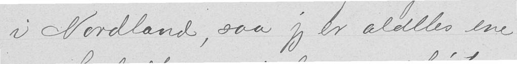i Nordland, saa jeg er aldeles ene
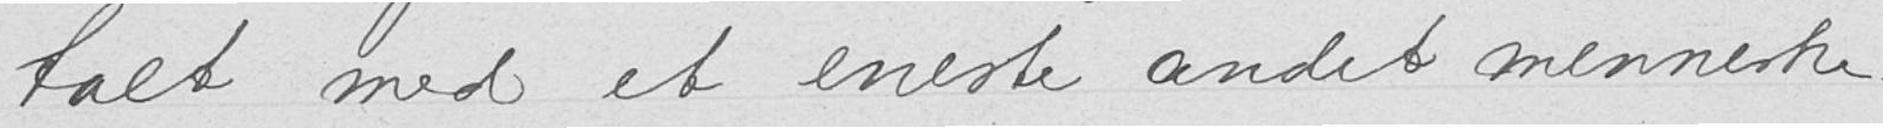talt med et eneste andet menneske.
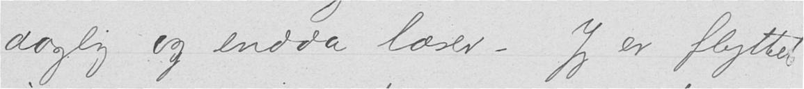daglig og endda læser – Jeg er flyttet
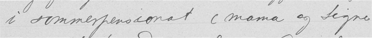i sommerpensionat (mama og Signe
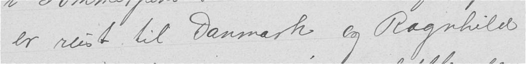er reist til Danmark og Ragnhild
